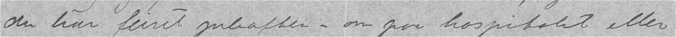du har feiret julaften — om paa hospitalet eller
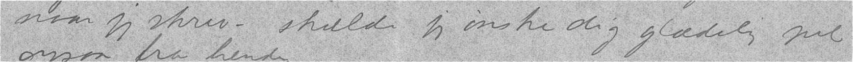naar jeg skrev — skulde jeg ønske dig glædelig
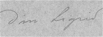Din Sigrid
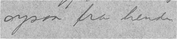jul ogsaa fra hende.
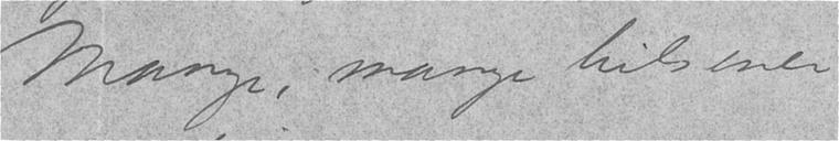Mange, mange hilsener
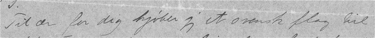Til ære for dig kjøber jeg et svensk flag
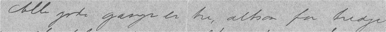Alle gode ganger er tre, altsaa for tredje
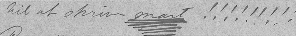til at skrive snart!!!!!!!!!
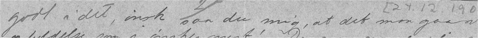godt i det, ønsk saa du mig, at det maa gaa i
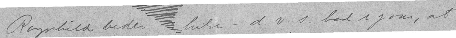Ragnhild beder hilse — d.v.s. bad igaar, at
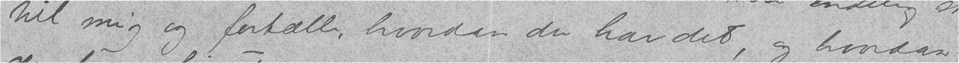til mig og fortælle, hvordan du har det, og hvordan
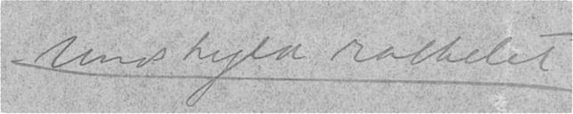Undskyld rabbelet
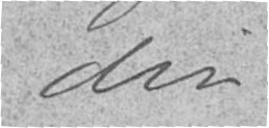din
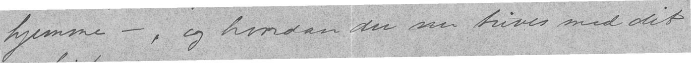hjemme —, og hvordan du nu trives med dit
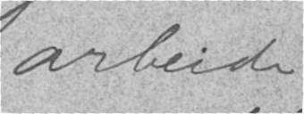arbeide.
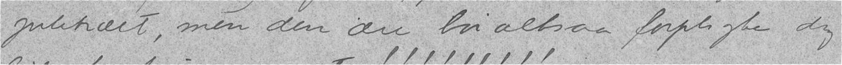til juletræet, men den ære bør altsaa forpligte dig
